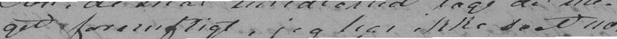get fornuftigt, jeg har ikke seet no-
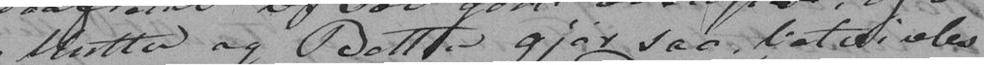Mutter og Betten gjør saa, betvivles
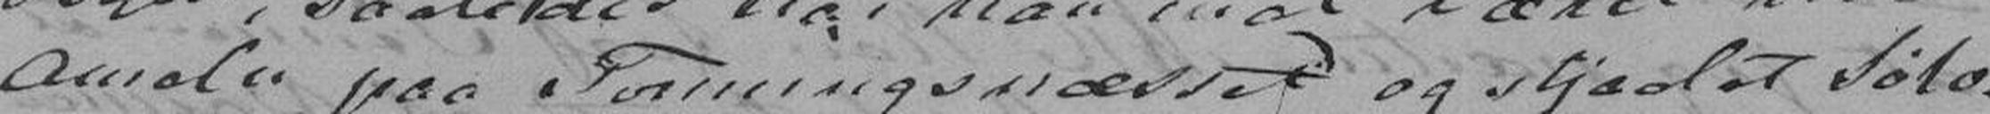Amalie paa Tonningnæsset og stjalet Sølv-
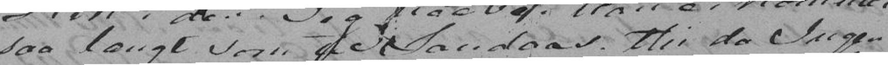saa langt som til Landaas, thi da Ingen
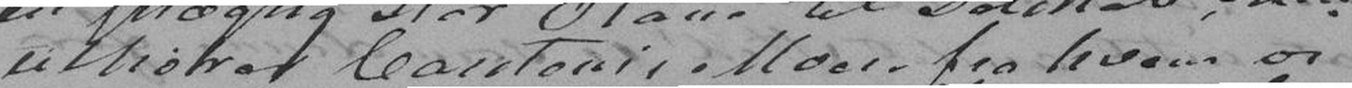tilhøre Carstens Moers fra hvem vi
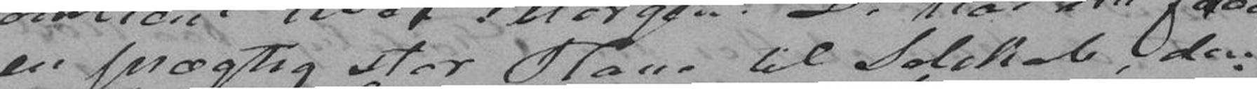en prægtig stor Hane til Selskab, den
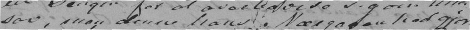sov, men denne hans Nærgaaenhed gjor-
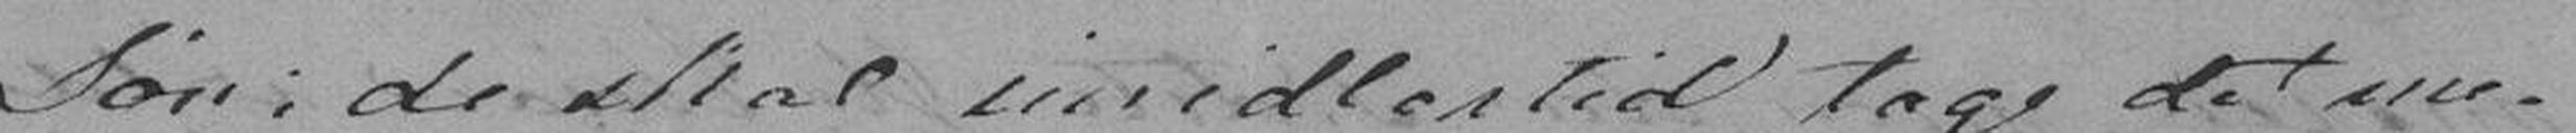Søn; de skal imidlertid tage det me-
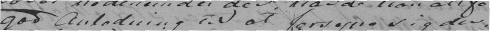god Anledning til at forsyne sig der
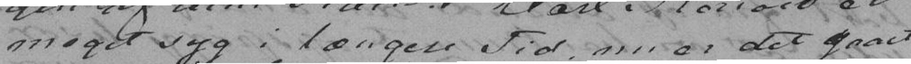meget syg i længere Tid, nu er det gaaet
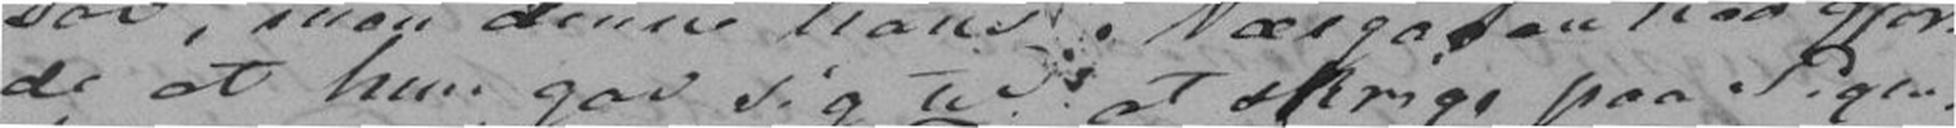de at hun gav sig til at skrige paa Pigen
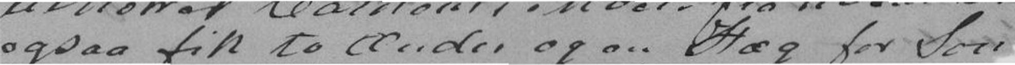ogsaa fik to Ænder og en Stæg for Som
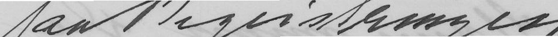faa Begeistringen
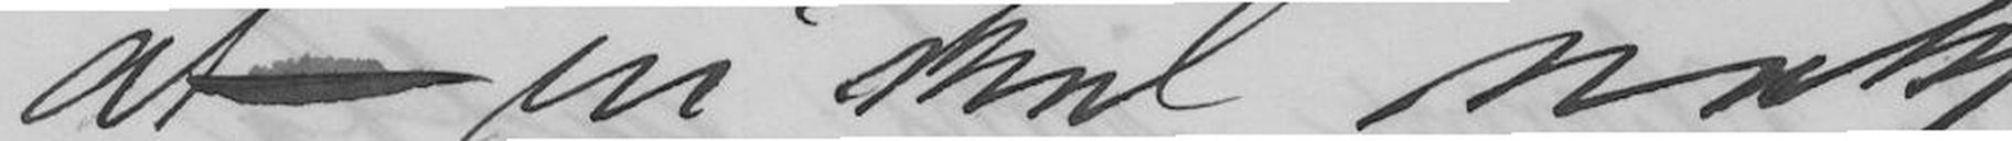at vi skal nok
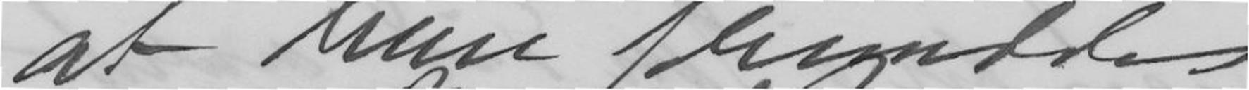at hun fremdeles
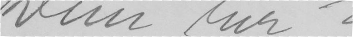Dem her?
